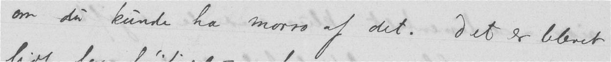om du kunde ha morro af det. Det er blevet
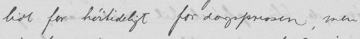lidt for høitideligt for dagspressen, men
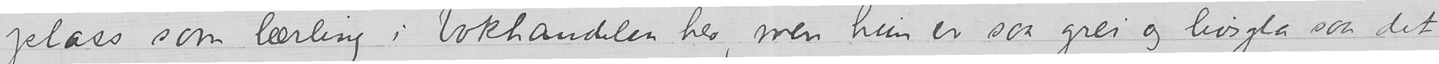plass som lærling i bokhandelen her, men hun er saa grei og livsgla saa det
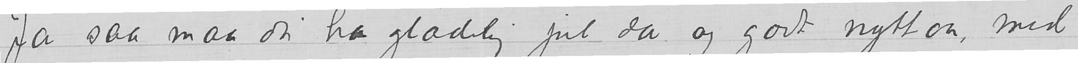Ja saa maa du ha glædelig jul da og godt nytt ar, med
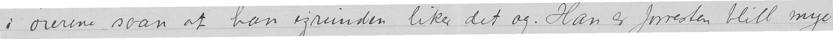i ørerne saan at han igrunden liker det og.  Han er forresten blitt mye
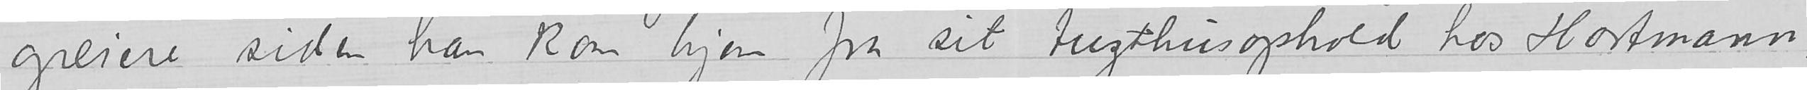greiere siden han kom hjem fra sit tugthusophold hos Hortmann,
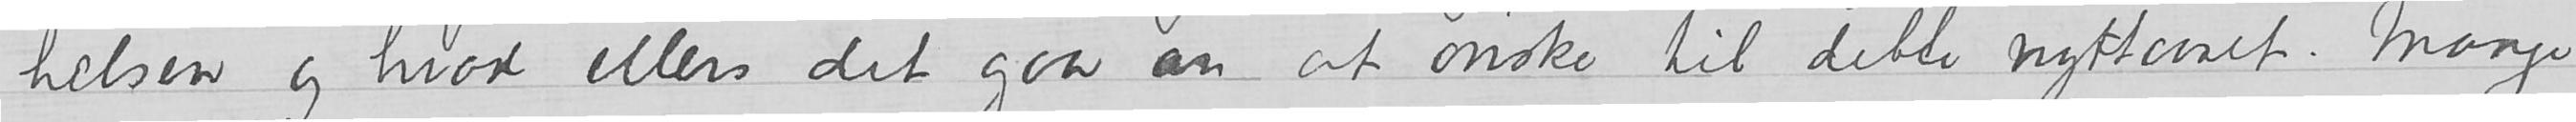helsen og hvor ellers det gar an at ønske til dette nyttaret. Mange
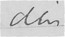din
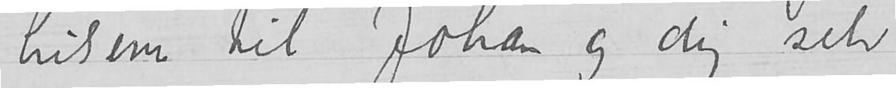hilsener til Johan og dig selv
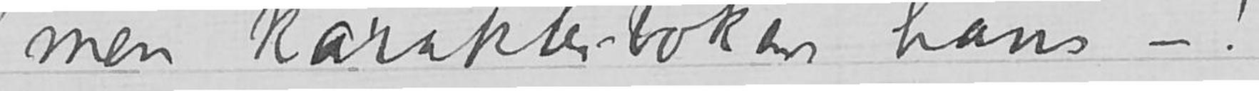men karakterboken hans - !
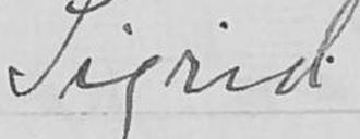Sigrid
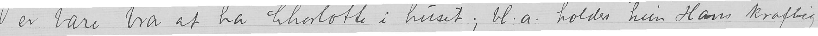er bare bra at ha Charlotte i huset; bl.a. holder hun Hans kraftig
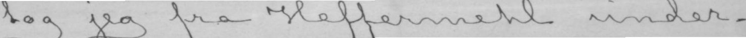tog jeg fra Heffermehl under-
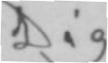Dig
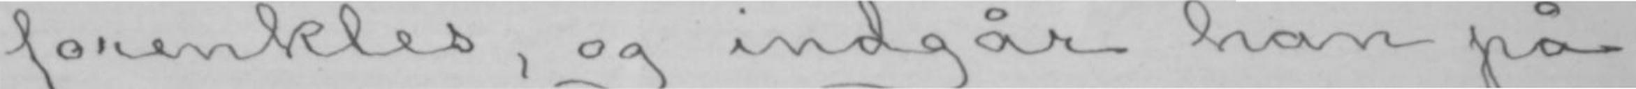forenkles, og indgår han på
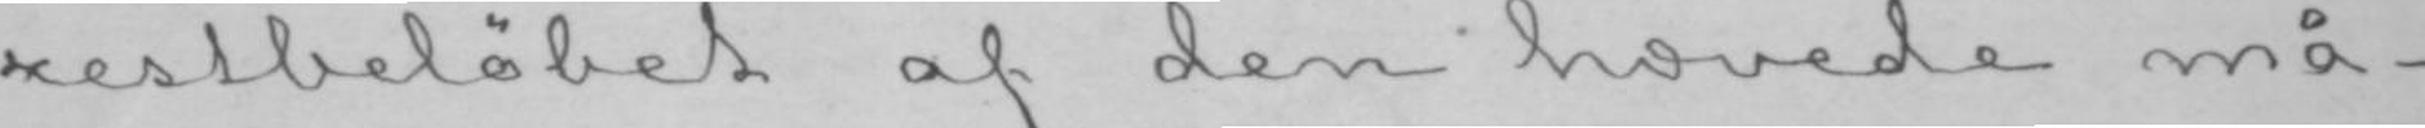restbeløbet af den hævede må-
